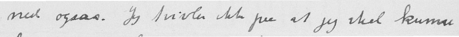ned ogsaa. Jeg tvivler ikke paa at jeg skal kunne
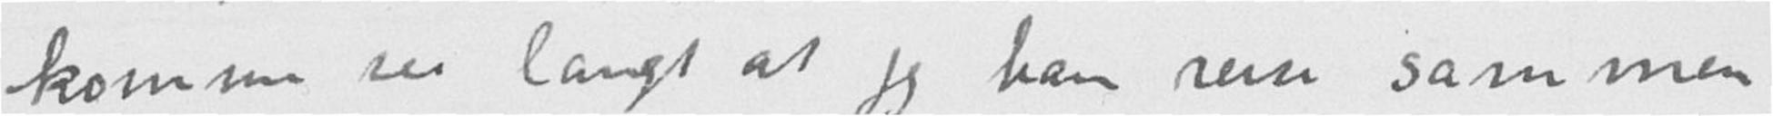komme saa langt at jeg kan reise sammen
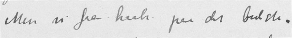Men vi faar haabe paa det bedste.
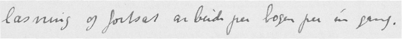læsning og fortsat arbeide paa bogen paa én gang.
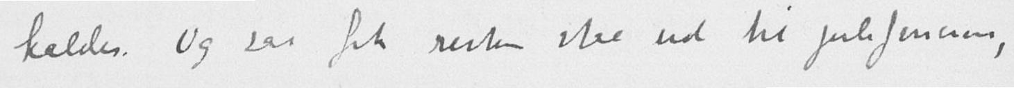kaldes. Og saa fik resten staa ud til juleferierne,
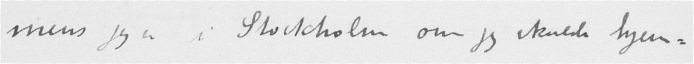mens jeg er i Stockholm om jeg skulde hjem-
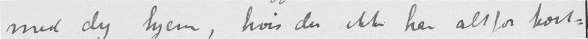med dig hjem, hvis du ikke har altfor kort-
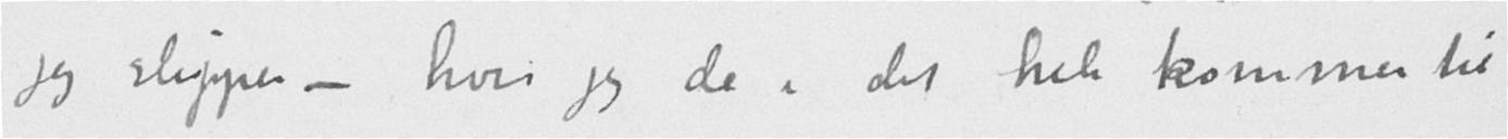jeg slipper - hvis jeg da i det hele kommer til
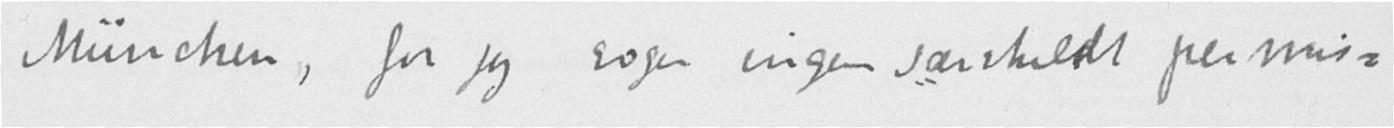München, for jeg søger ingen særskildt permis-
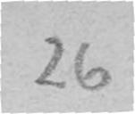26
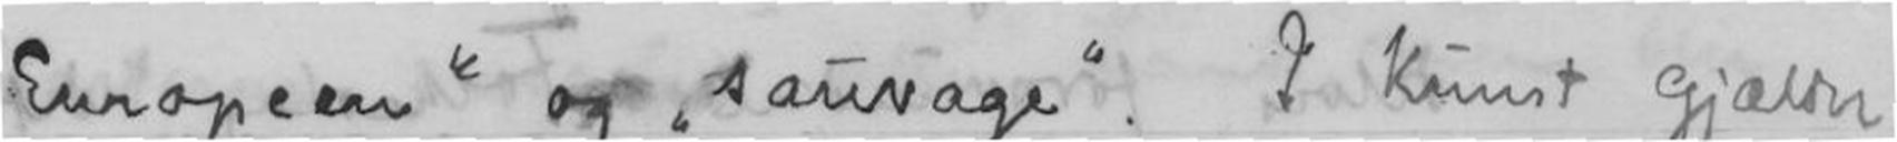Europeen og sauvage. I kunst gjælder
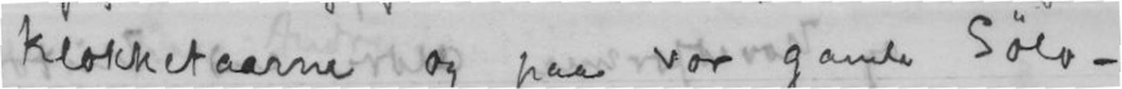klokketaarne og paa vor gamle Sølv-
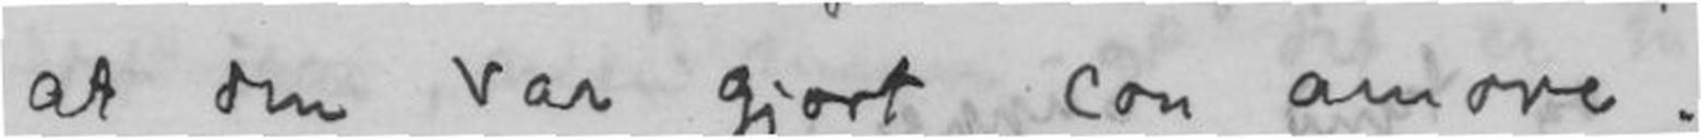at den var gjort con amore.
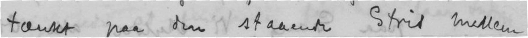tænkt paa den staaende Strid mellem
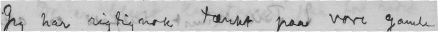Jeg har rigtignok tænkt paa vore gamle
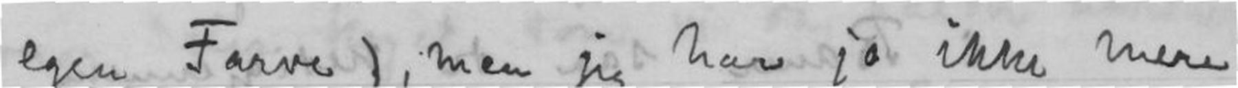egen Farve), men jeg har jo ikke mere
